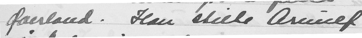Øverland. Han stilte Arnulf
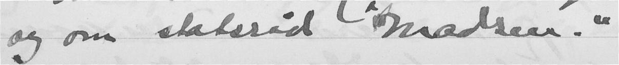og om statsråd Madsen."
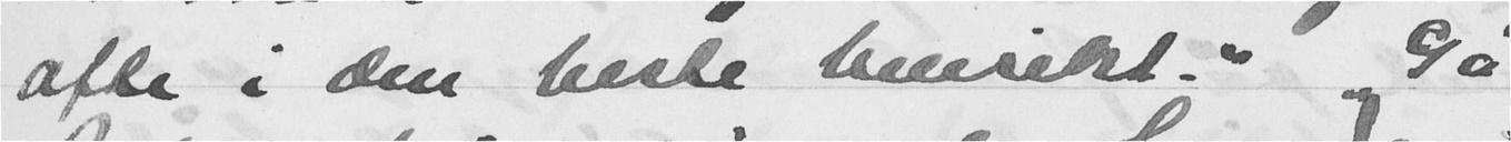ofte i den beste hensikt." Gå
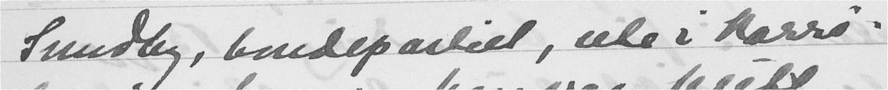Sundby, bondepartiet, ute i korri-
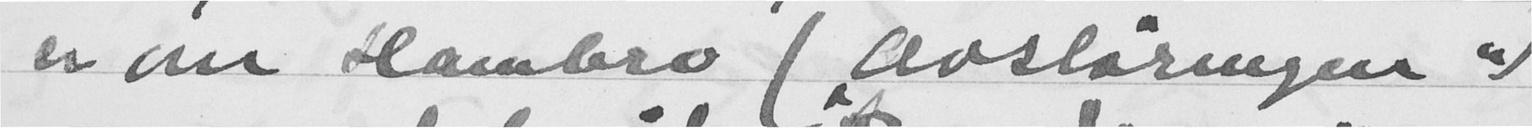er om Hambro ("Avsløringen")
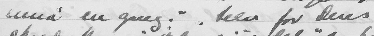ennå en gang." "Selv for Deres
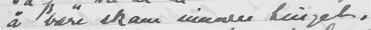å bære skam innover bruget,
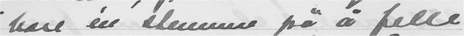bare en stemme på å felle
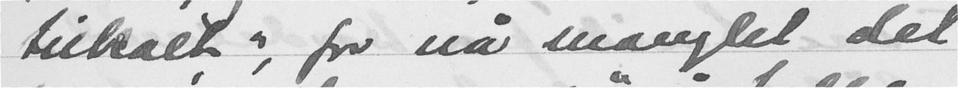"tiltalt", for nå manglet det
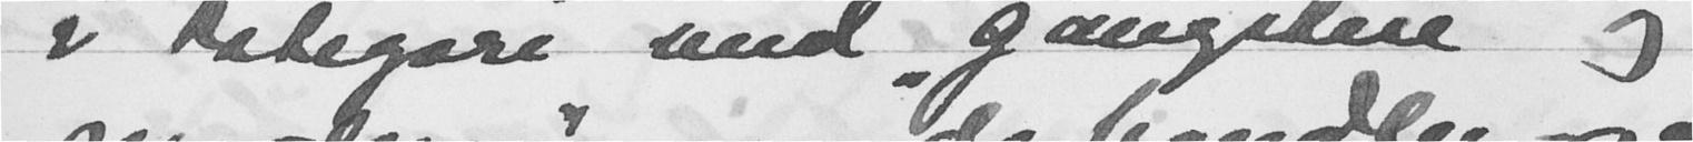i kategori med "gangstere og
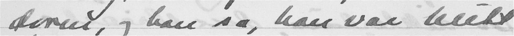doren, og han sa, han var blitt
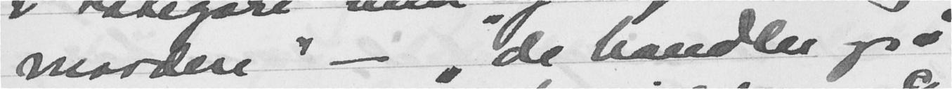mordere" - "de handler også
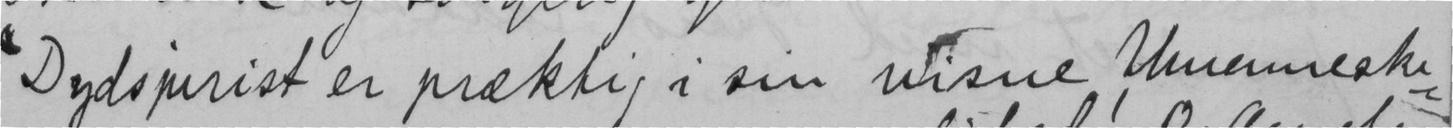Dydsjurist er præktig i sin visne Umenneske-
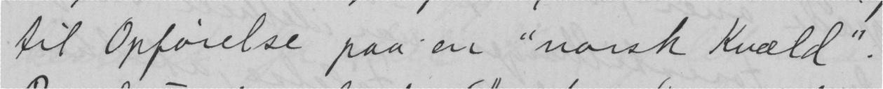til Opførelse paa en "norsk Kvæld".
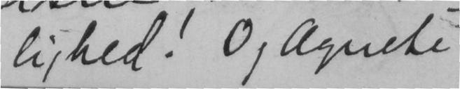lighed! Og Agnete
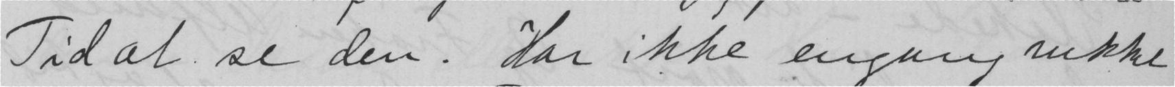Tid at se den. Har ikke engang rukke
